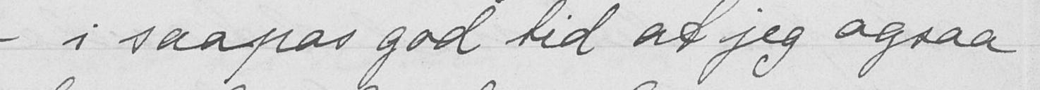- i saapas god tid at jeg ogsaa
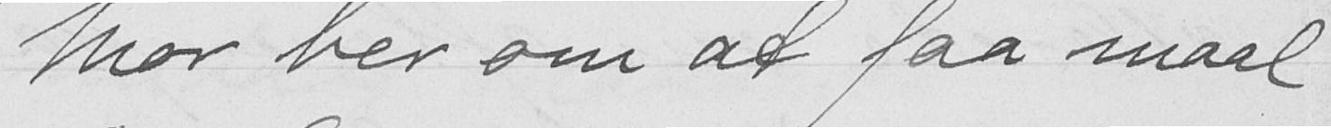Mor ber om at faa maal
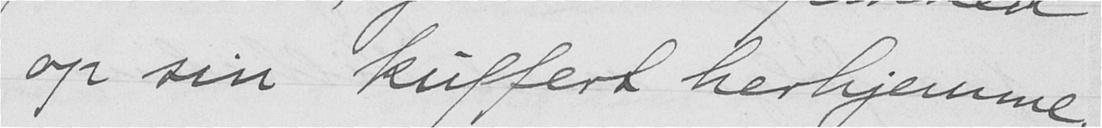op sin kuffert herhjemme,
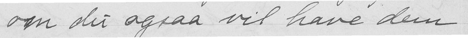om du ogsaa vil have dem
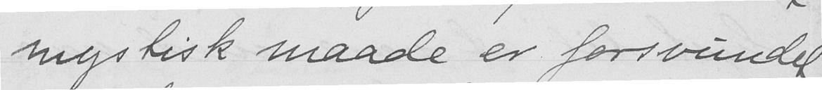mystisk maade er forsvundet
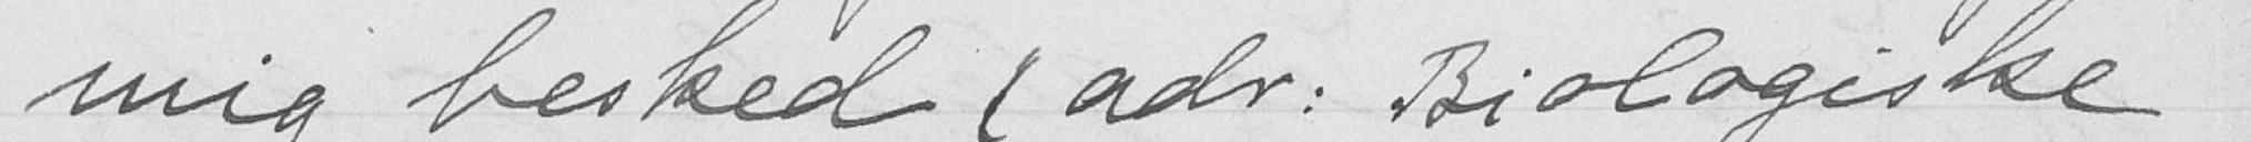mig besked (adr. Biologiske
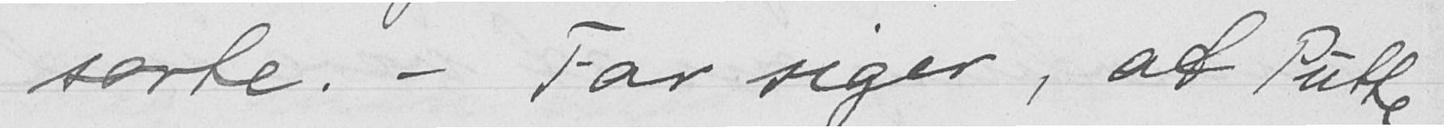sorte. - Far siger, at Putte
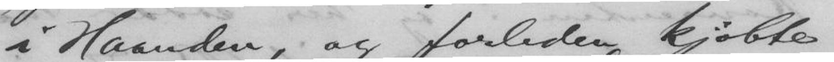i Haanden, og forleden kjøbte
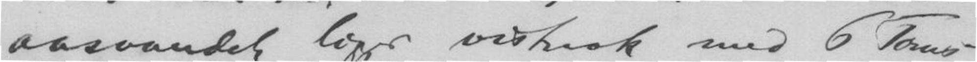aasvandet ligger vistnok med 6 Toms
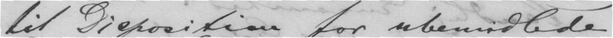til Disposition for ubemidlede
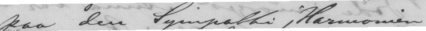paa den Sympathi, "Harmonien"
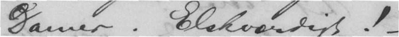Damer. Elskværdigt! -
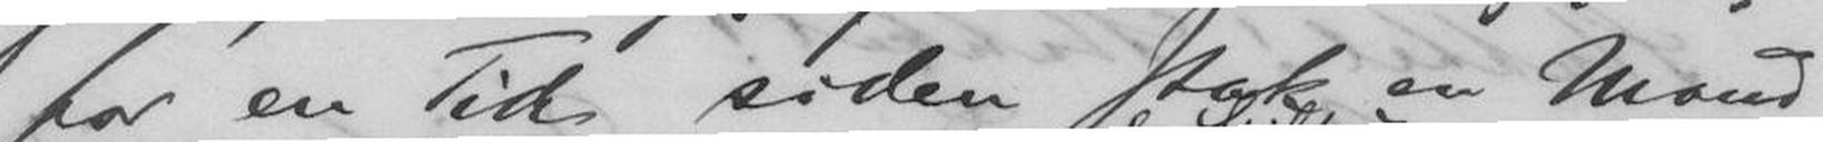for en Tid siden stak en Mand
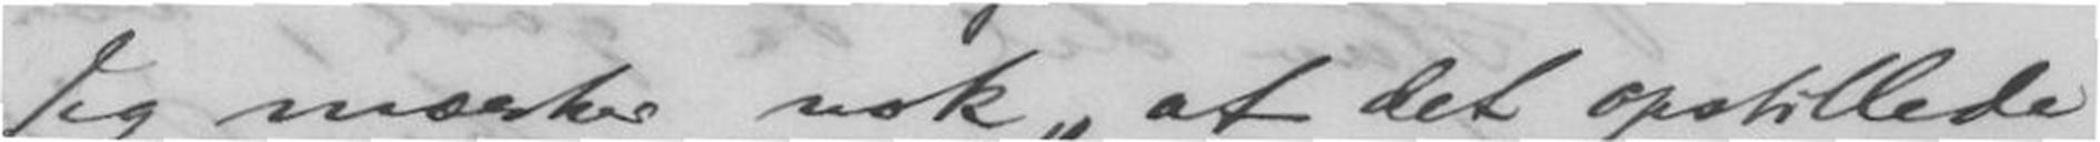Jeg mærker nok, at det opstillede
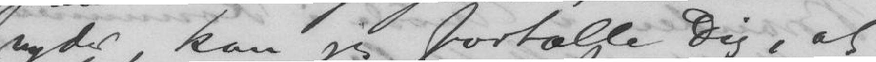nyder, kan jeg fortælle Dig, at
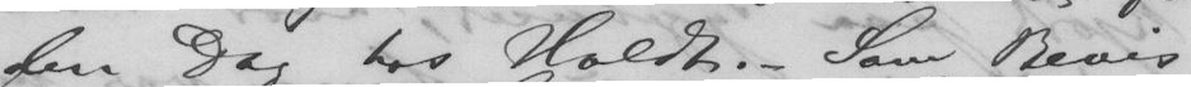den Dag hos Holdt. - Som Bevis
